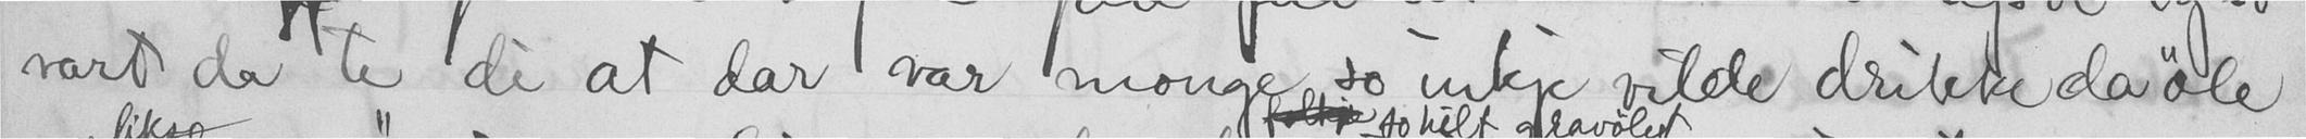vart da te di at dar var monge so inkje vilde drikke da øle
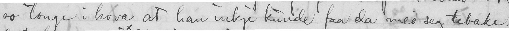so tonge i hova at han inkje kunde faa da med seg tebake.
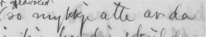so mykkje atte av da
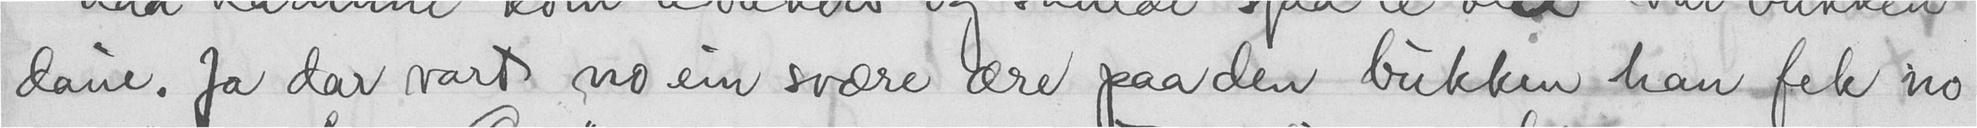daue. Ja dar vart no ein svære ære paa den bukken han fek no
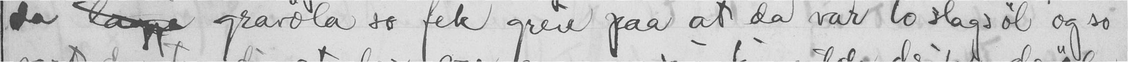da  gravøla so fek greie paa at da var to slags øl og so
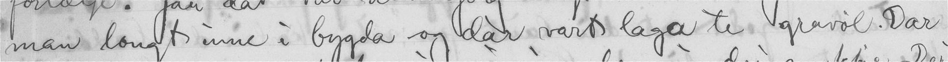man longt inne i bygda og dar vart laga te gravøl. Dar
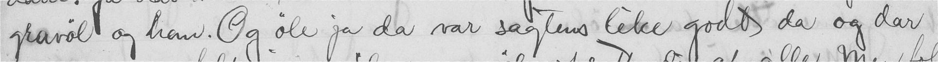gravøl og han. Og øle ja da var sagtens like godt da og dar
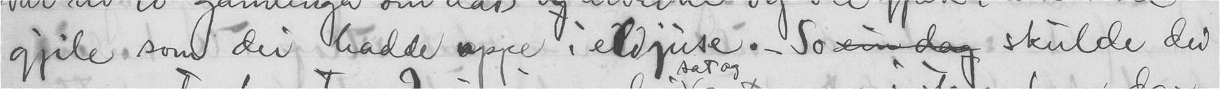gjile som dei hadde oppe i eldjuse. - So  skulde dei
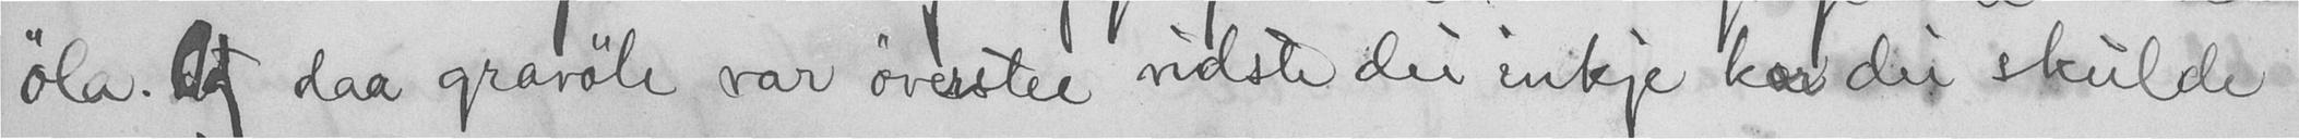øla. Og daa gravøle var øverstee vidste dei inkje kor dei skulde
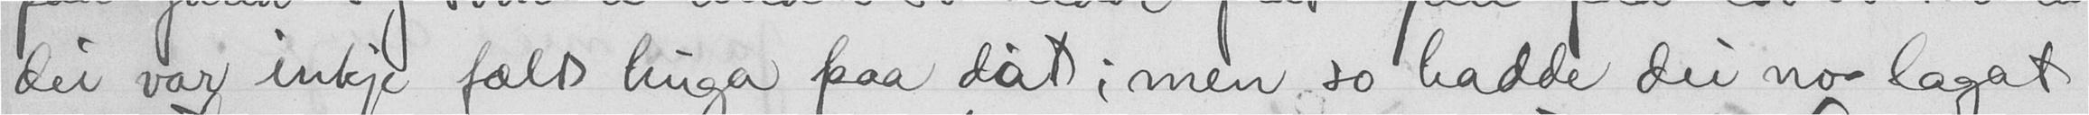dei var inkje fælt huga paa dat; men so hadde dei no lagat
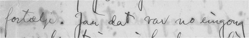fortælje. Jau dat var no eingang
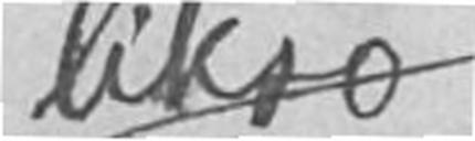likso
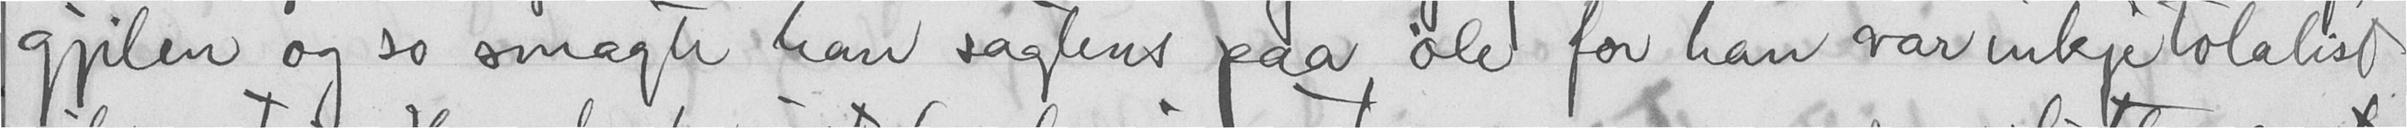gjilen og so smagte han sagtens paa øle for han var inkje tolalist
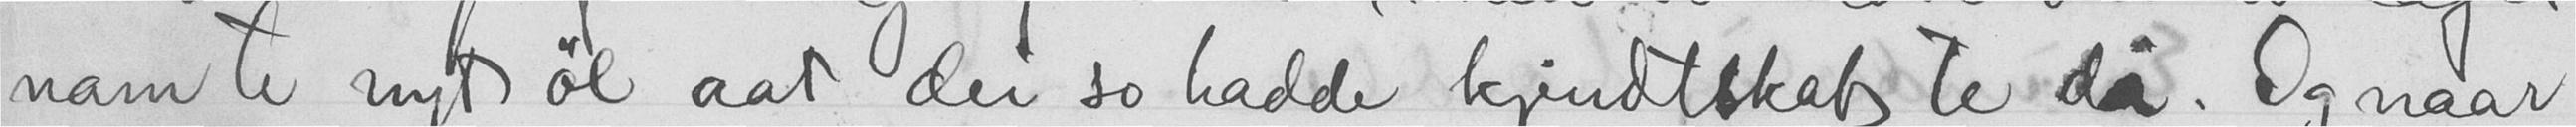nam te nyt øl aat dei so hadde kjendtskab te då. Og naar
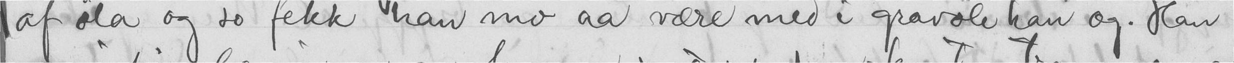af øla og so fekk han mo aa være med i gravøle han og. Han
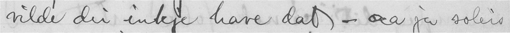vilde dei inkje have dat - aa ja soleis
